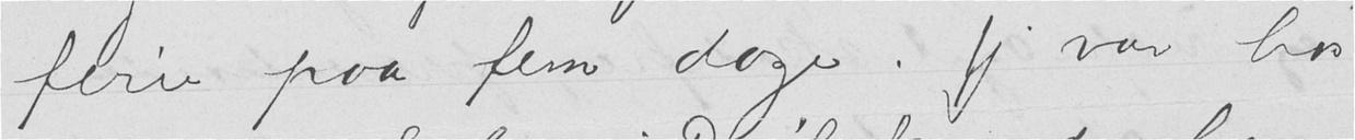ferie paa fem dage. Jeg var hos
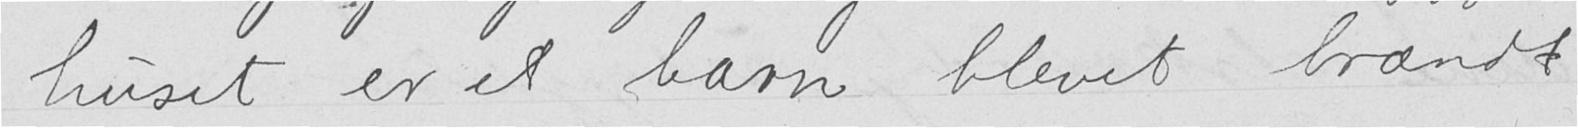huset er et barn blevet brændt
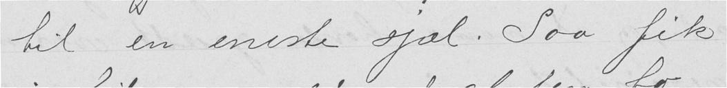til en eneste sjæl. Saa fik
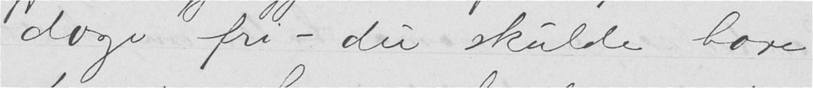dage fri – du skulde bare
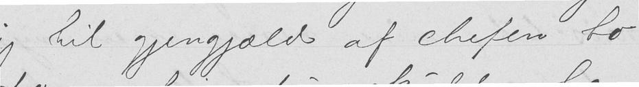jeg til gjengjeld af chefen to
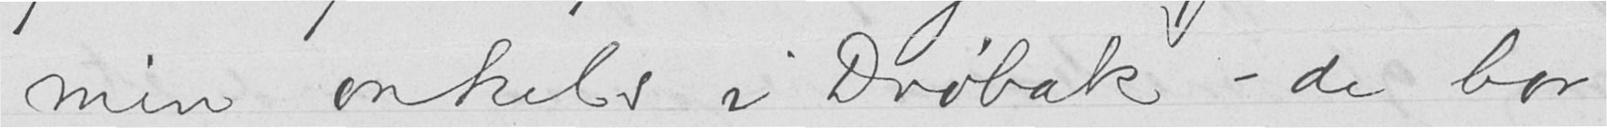min onkels i Drøbak - de bor
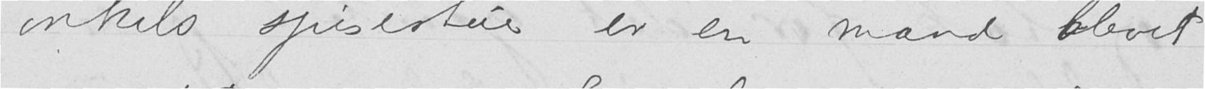onkels spisestue er en mand blevet
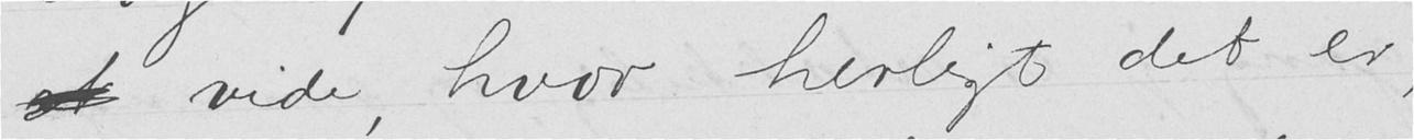vide, hvor herligt det er,
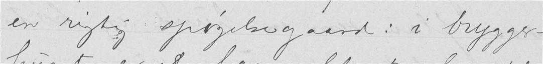en rigtig spøgelsesgaard: i brygger-
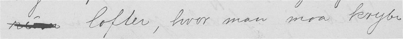løfter, hvor man maa krybe
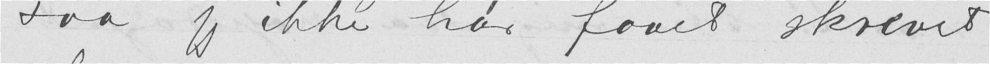saa jeg ikke har faaet skrevet
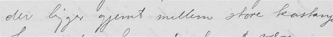der ligger gjemt mellem store kastanje-
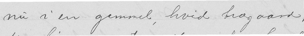nu i en gammel, hvid trægaard,
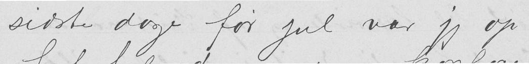sidste dager før jul var jeg op-
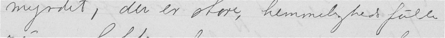myrdet; der er store, hemmelighedsfulde
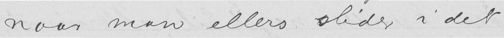naar man ellers slider i det
